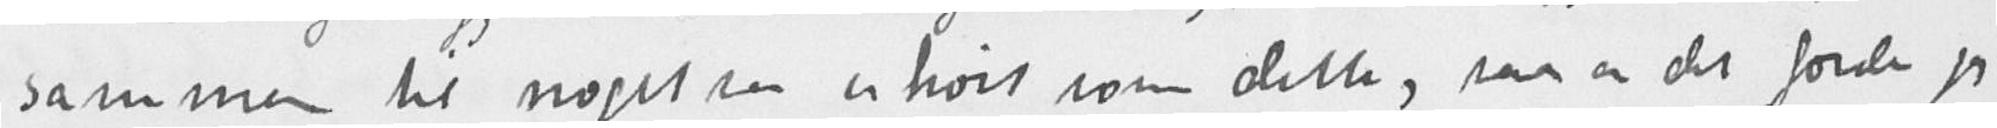sammen til noget saa erhøit som dette, saa er det fordi jeg
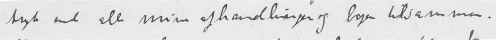tryk end alle mine afhandlinger og bøger tilsammen.
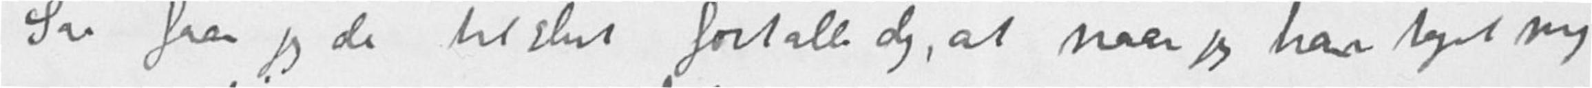Saa faar jeg da tilslut fortælle dig, at naar jeg har taget mig
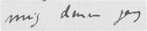mig denne gang
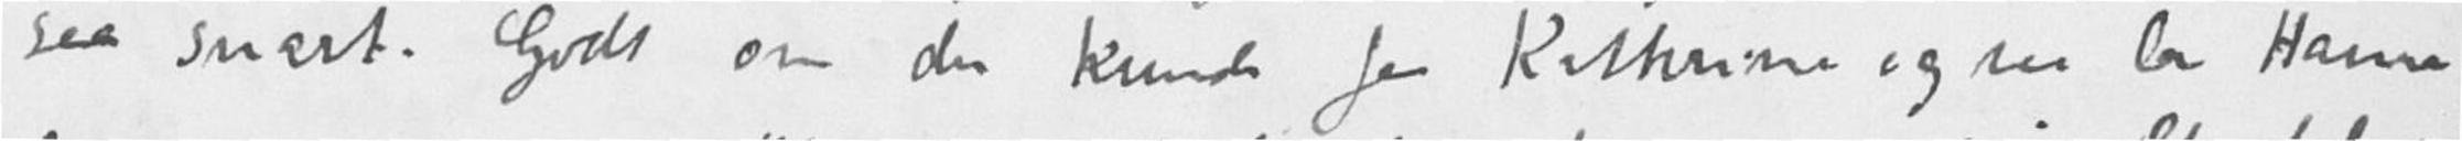saa snart. Godt om du kunde faa Kathrine og saa la Hanna
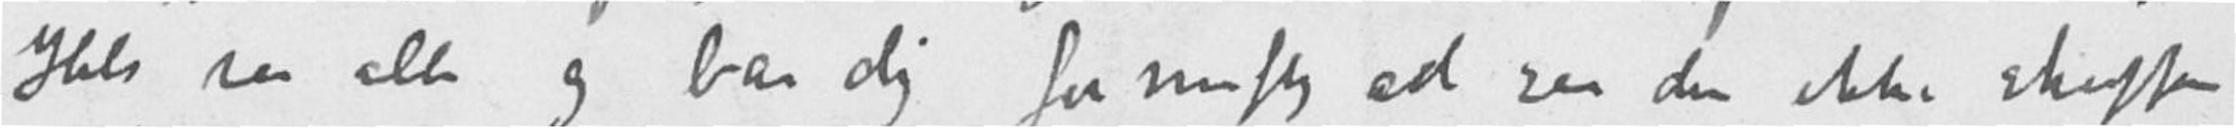Hils saa alle og bær dig fornuftig ad saa du ikke skuffer
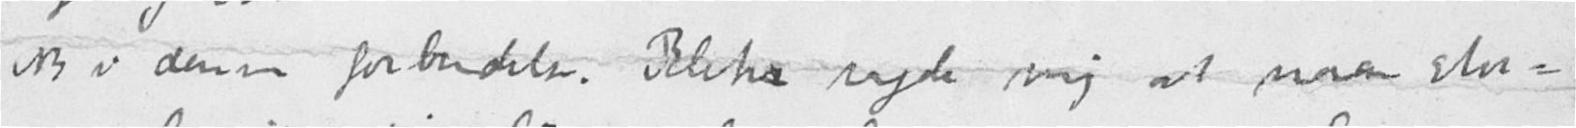NB i denne forbindelse. Blihe sagde mig at naar stor-
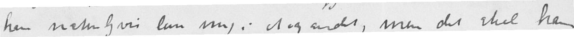han naturligvis lure mig i et og andet, men det skal han
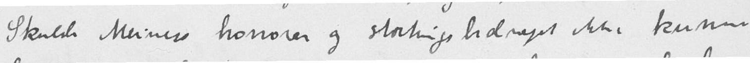Skulde Meiners honorar og stortingsbidraget ikke kunne
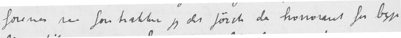forenes saa foretrækker jeg det første da honoraret for begge
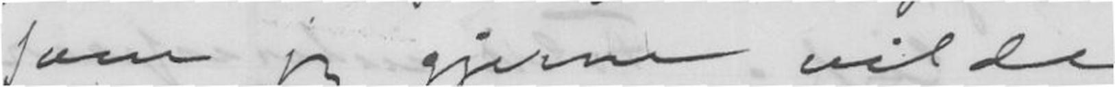som jeg gjerne vilde
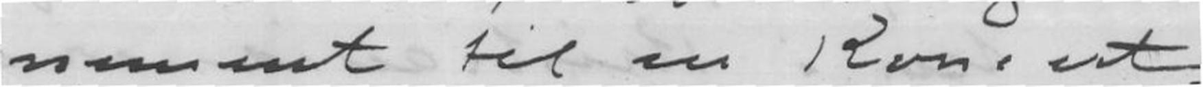nement til en koncert.
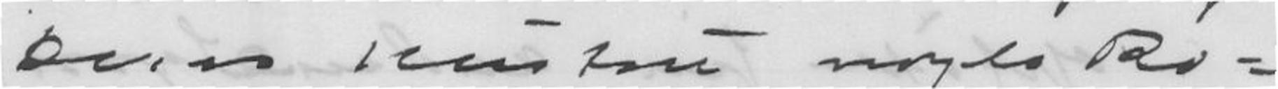Deres Hustru nogle Ro-
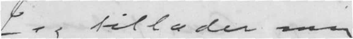Jeg tillader mig
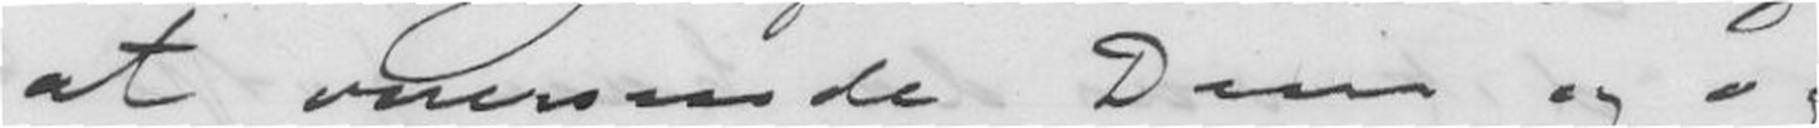at oversende Dem og og
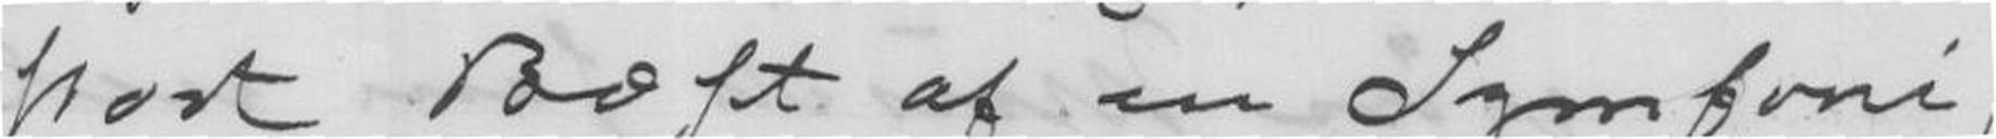stort Bæst af en Symfoni,
